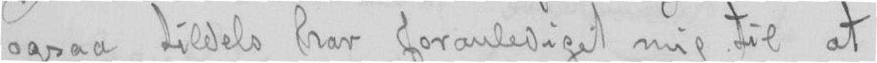ogsaa tildels har foranlediget mig til at
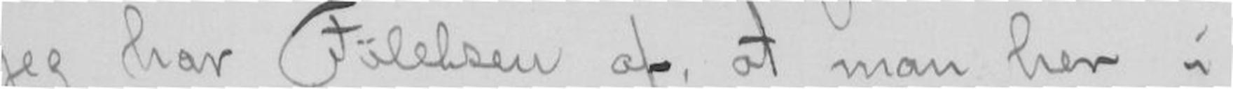jeg har Følelsen af, at man her i
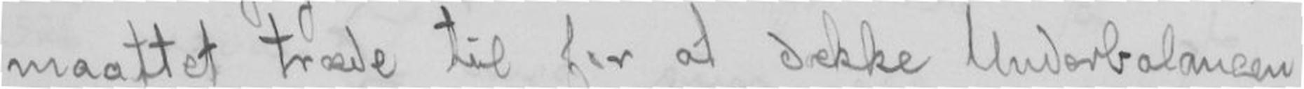maattet træde til for at dekke Underbalancen
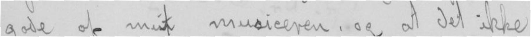gode af mit musiceren, og at det
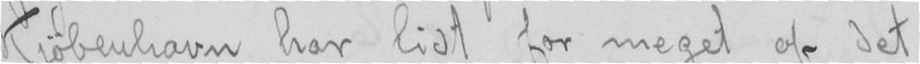Kjøbenhavn har lidt for meget af det
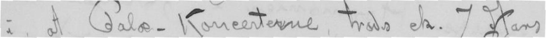i at Palæ-Koncerterne trods ek. 7 Aars
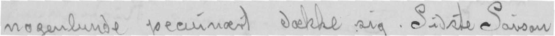nogenlunde pecuinært dække sig. Sidste Saison
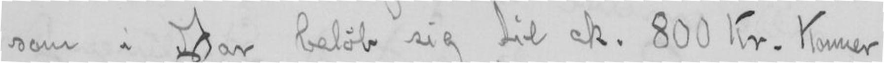som i Aar beløb sig til ck. 800 Kr. Komer nu
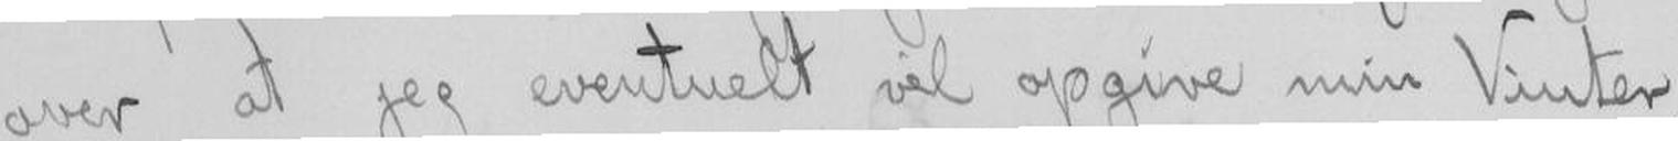over at jeg eventuelt vil opgive min Vinter-
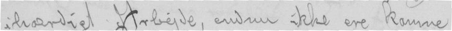ihærdigt Arbeide, endnu ikke ere komne
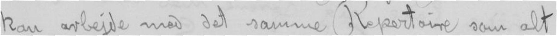kan arbejde med det samme Repertoire som alt
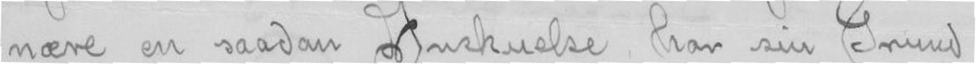nære en saadan Anskuelse har sin Grund
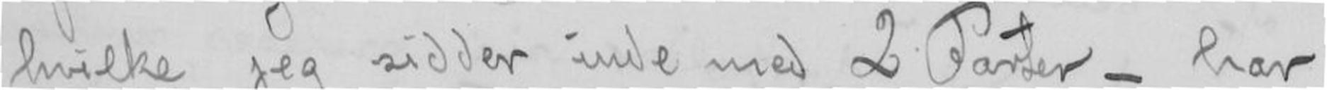hvilke jeg sidder inde med 2 Parter - har
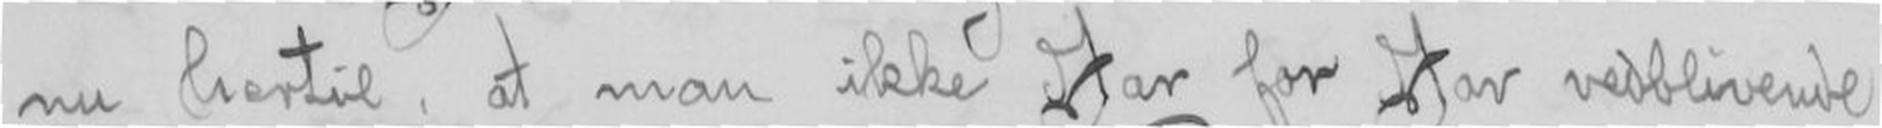hertil at man ikke Aar for Aar vedblivende
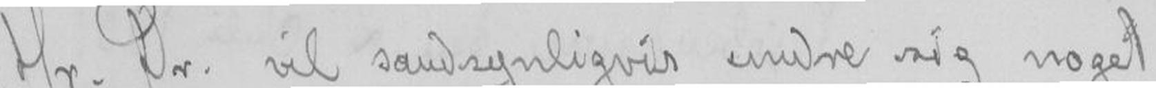Hr. Dr. vil sandsynligvis undre sig noget
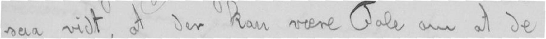saa vidt, at der kan være Tale om at de
